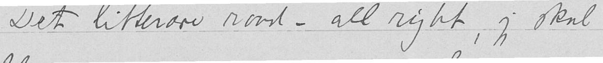Det litterære raad – all right, jeg skal
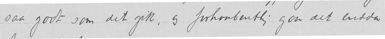saa godt som det gik, og forhaabentlig gaar det endda
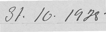31.10.1928.
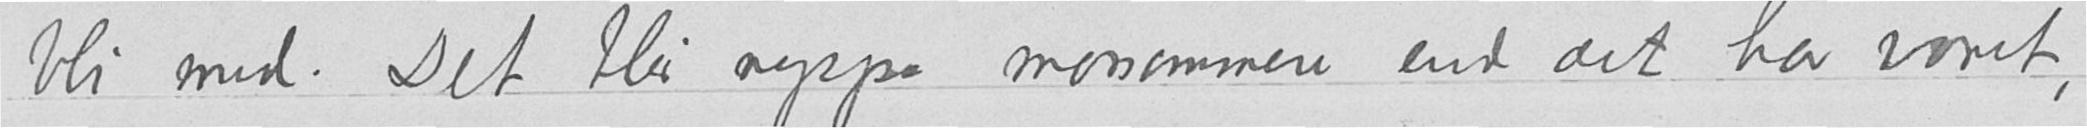bli med. Det blir neppe morsommere end det har været,
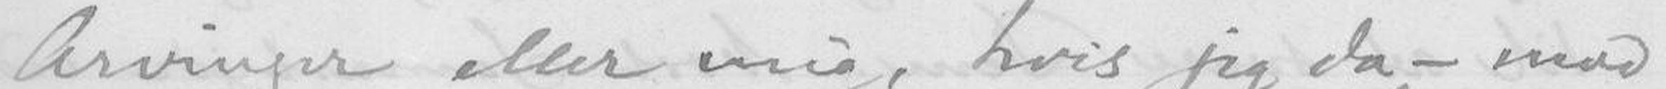Arvinger eller mig, hvis jeg da - mod
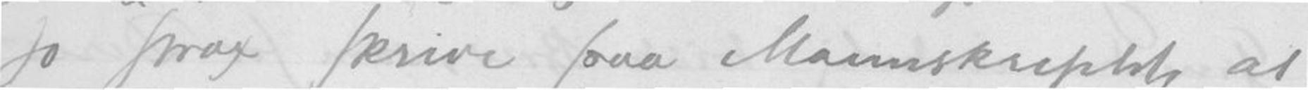jo strax skrive paa Manuskriphte at
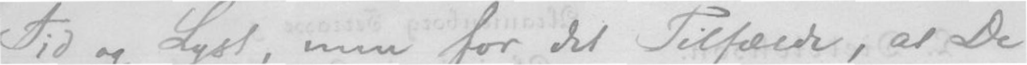Tid og lyst, men for det Tilfælde, at De
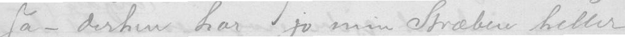Ja - derhen har jo min Stræben heller
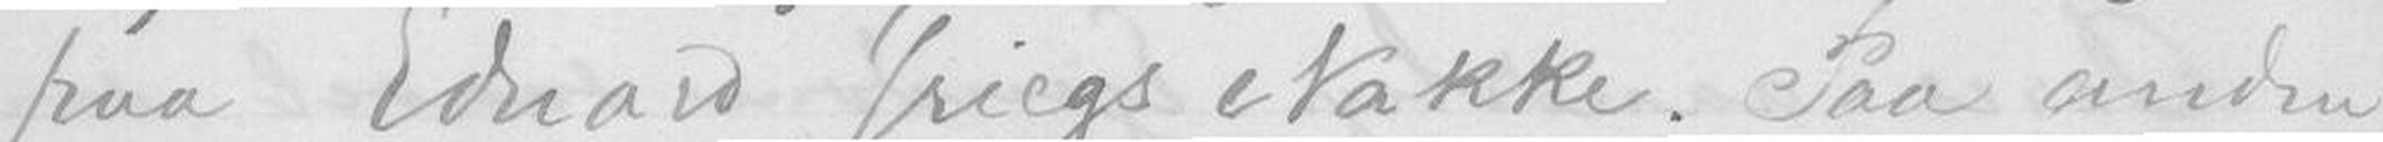paa Edvard Griegs Nakke. Paa anden
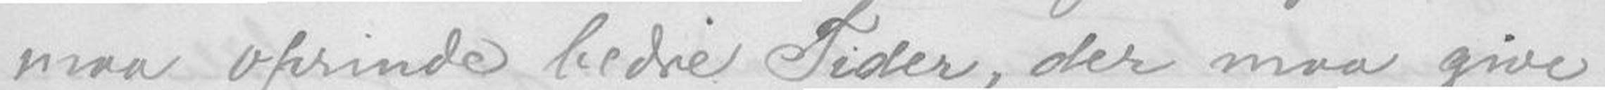maa oprinde bedre Tider, der maa give
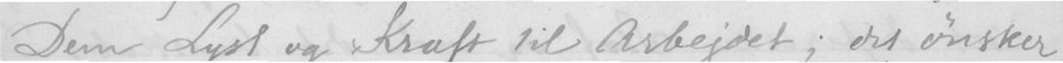Dem Lyst og Kraft til Arbejdet; det ønsker
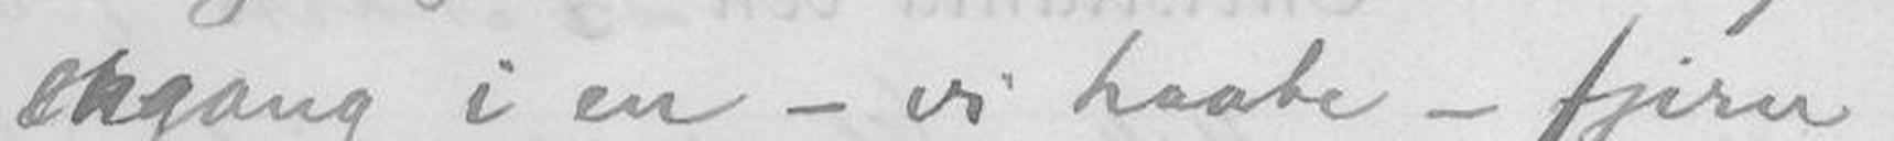engang i en - vi haabe - fjern Fremtid
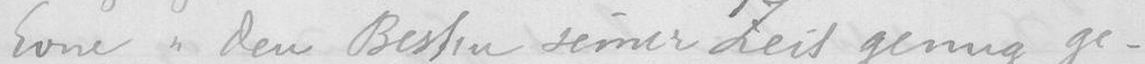Evne den Besten simer Zeit genug ge-
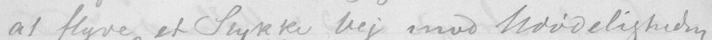at flyve et Stykke vej mod Udødeligheden
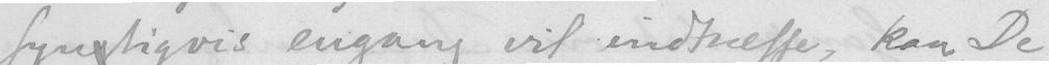syngligvis engang vil indtræffe, kan De
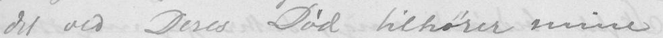det ved Deres Død tilhører mine
